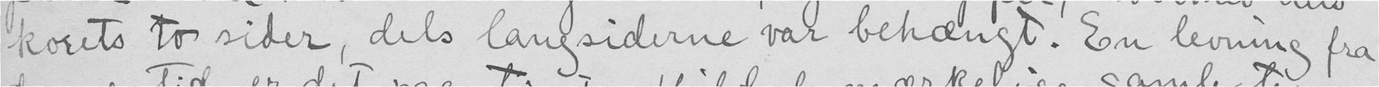korets to sider, dels langsiderne var behængt. En levning fra
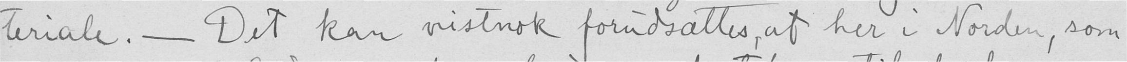teriale. - Det kan vistnok forudsættes, at her i Norden, som
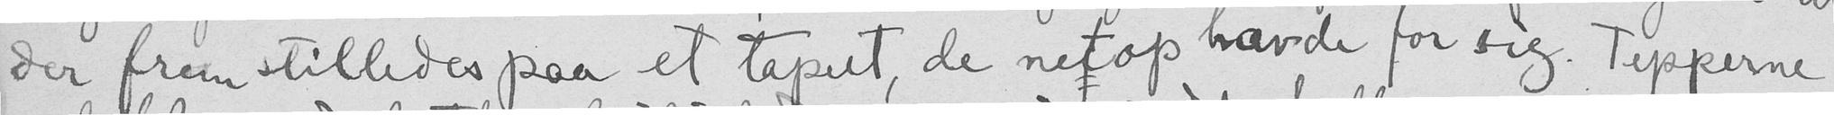der fremstilledes paa et tapet, de netop havde for sig. Tepperne
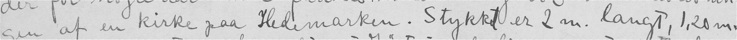en af en kirke paa Hedemarken. Stykket er 2 m. langt, 1,20 m.
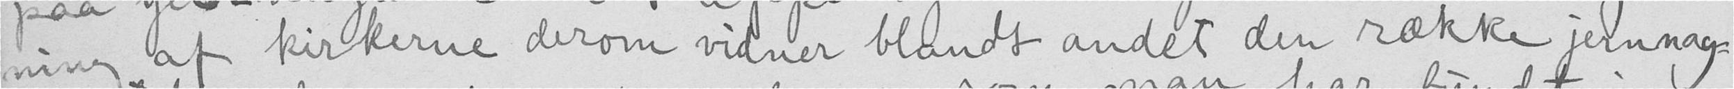ning af kirkerne derom vidner blandt andet den række jernnag-
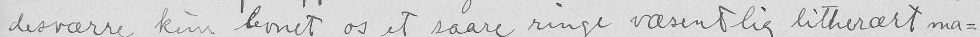desværre kun levnet os et saare ringe væsentlig litherært ma-
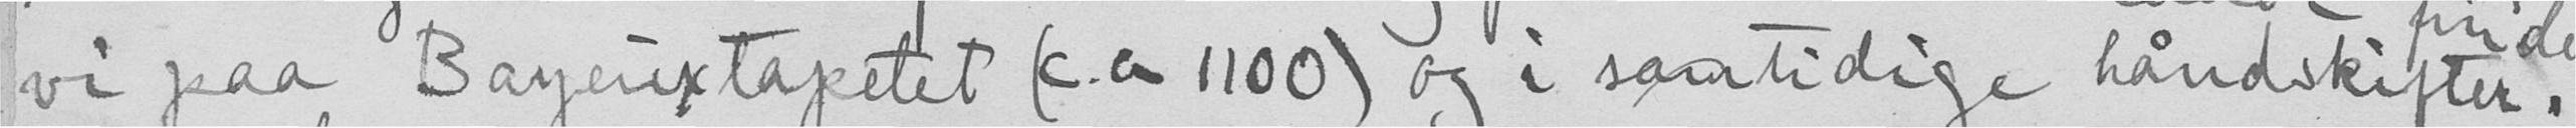vi paa Bayeuxtapetet (ca 1100) og i saatidige håndskifter.
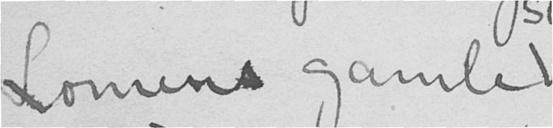Lomens gamle
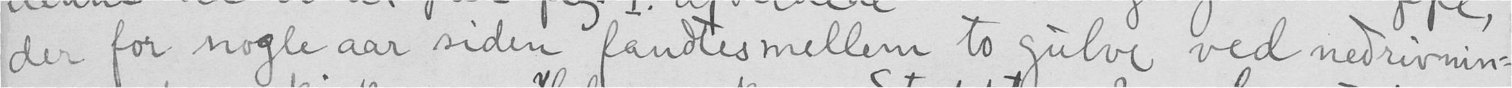der for nogle aar siden fandtes mellem to gulve ved nedrivnin-
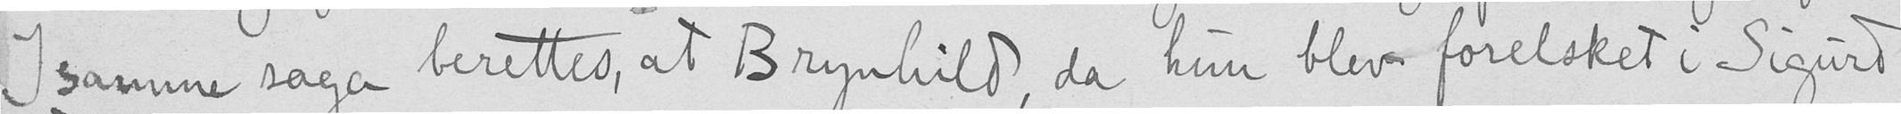I samme saga berettes, at Brynhild, da hun blev forelsket i Sigurd
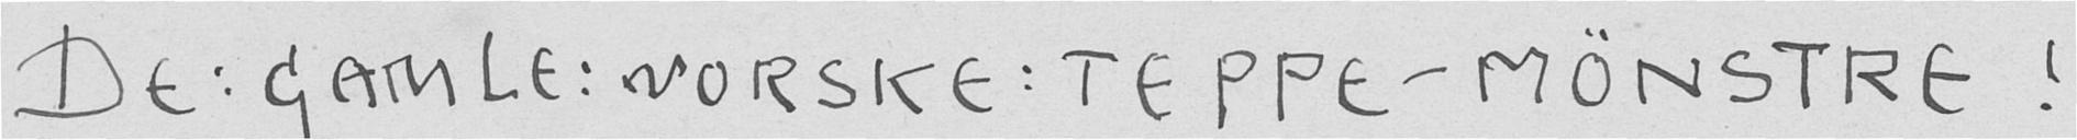DE: GAMLE: NORSKE: TEPPE-MØNSTRE!
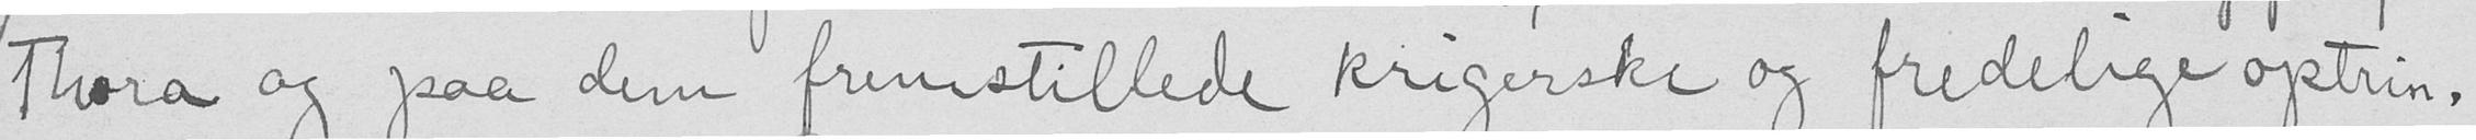Thora og paa dem fremstillede krigerske og fredelige optrin.
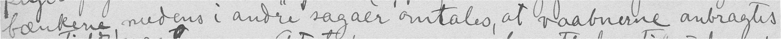bænkene, medens i andre sagaer omtales, at vaabnerne anbragtes
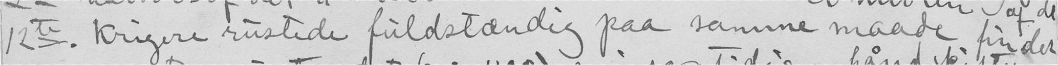12te. Krigere rustede fuldstændig paa samme maade finder
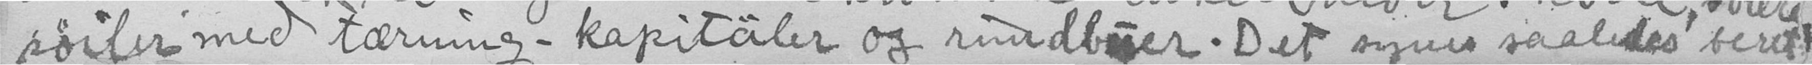søiler med tærning - kapitäler og rundbuer. Det synes saaledes beret
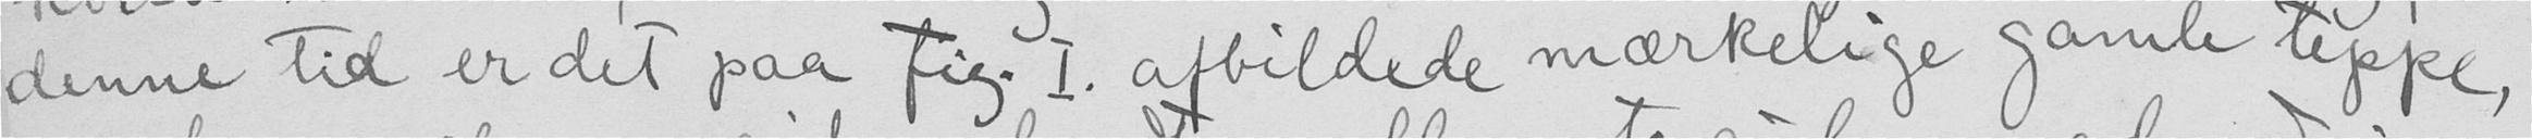denne tid er det paa fig. I. afbildede mærkelige gamle teppe,
